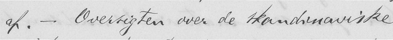af. - Oversigten over de skandinaviske
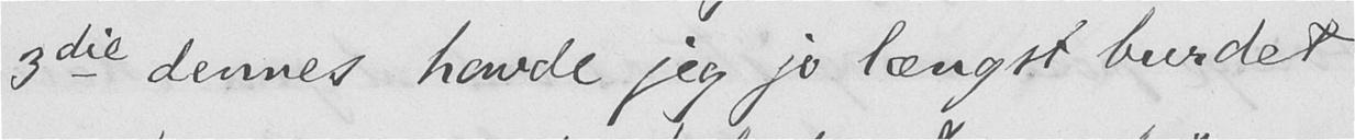3die dennes havde jeg jo længst burdet
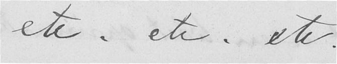etc. etc. etc.
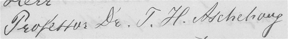Professor Dr. T. H. Aschehoug.
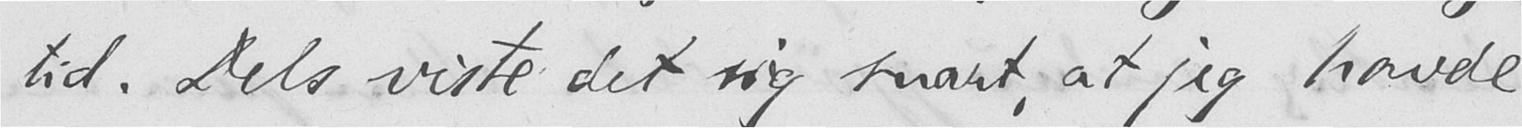tid. Dels viste det sig snart, at jeg havde
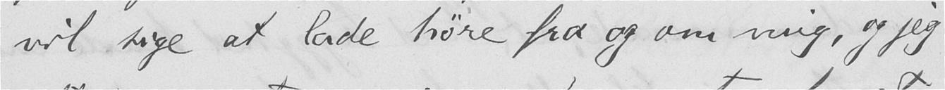vil sige at lade høre fra og om mig, og jeg
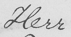Herr
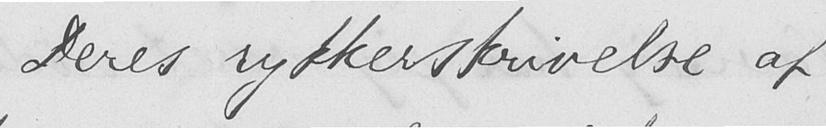Deres rykkerskrivelse af
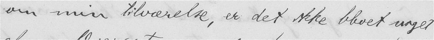om min tilværelse, er det ikke blivet noget
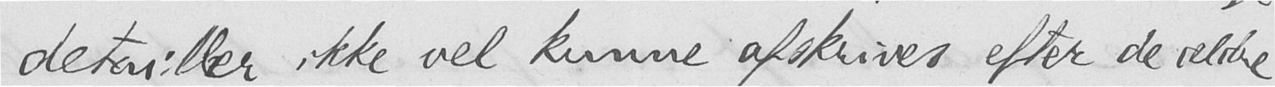detailler ikke vel kunne afskrives efter de ældre
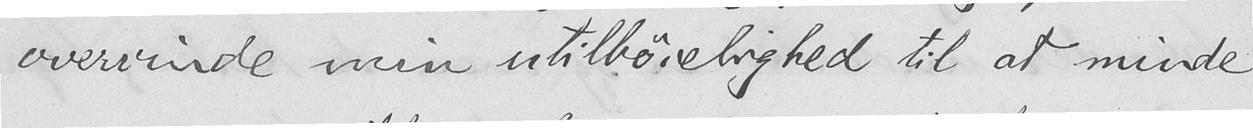overvinde min utilbøielighed til at minde
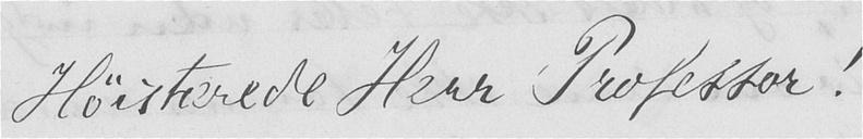Høistærede Herr Professor!
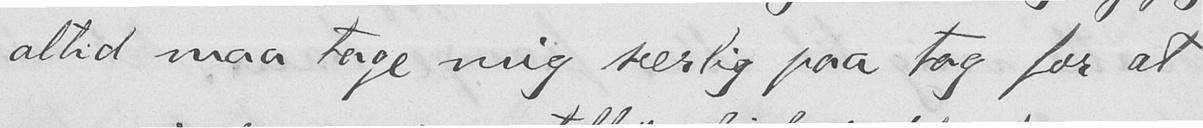altid maa tage mig særlig paa tag for at
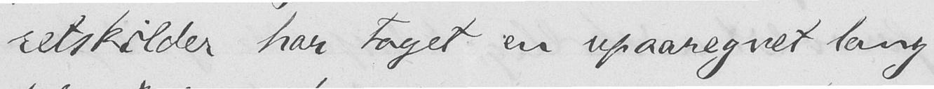retskilder har taget en upaaregnet lang
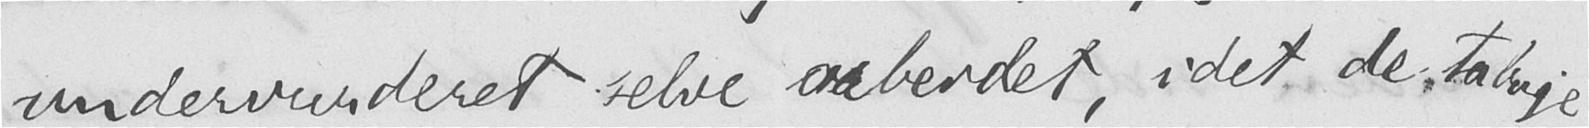undervurderet selve arbeidet, idet de talrige
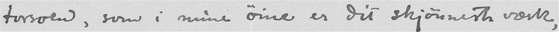torsoen, som i mine øine er dit skjønneste værk,
